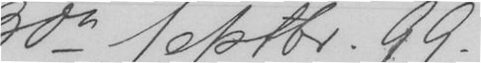30de Septbr. 99.
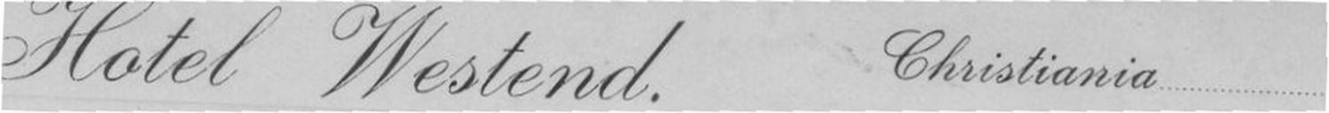Hotel Westend. Christiania
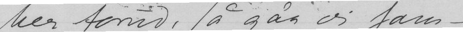her forud, så går vi sam-
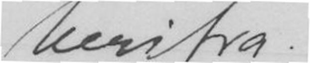herifra.
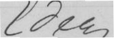Eders
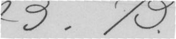B.B.
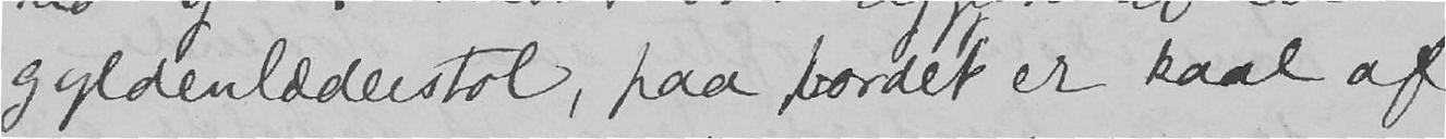gyldenlæderstol, paa bordet er kaal af
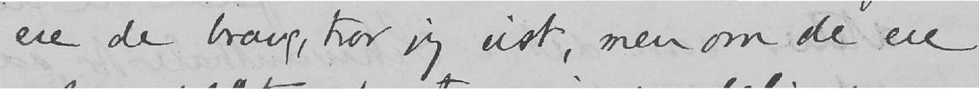ere de brave, tror jeg vist, men om de ere
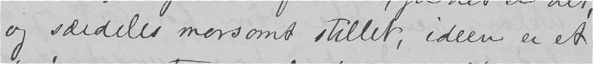og særdeles morsomt stillet, ideen er et
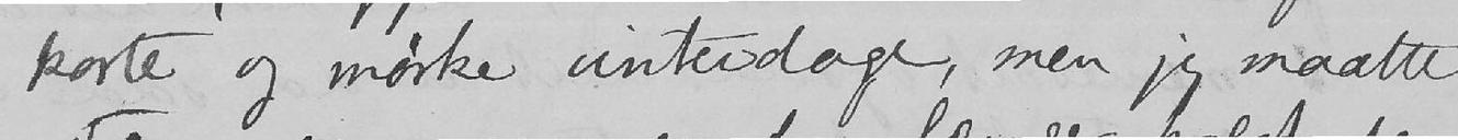korte og mørke vinterdage, men jeg maatte
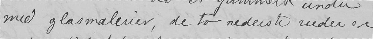med glasmalerier, de to nederste ruder ere
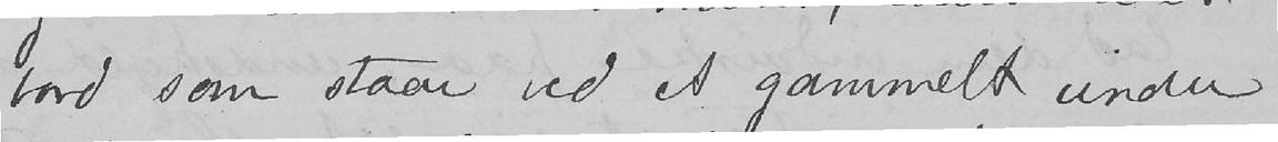bord som staar ved et gammelt vindu
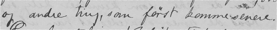og andre ting, som først komme senere.
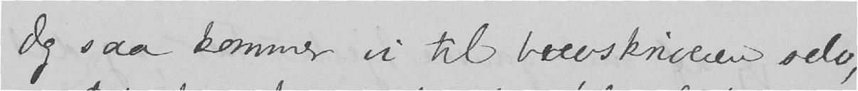Og saa kommer vi til brevskriveren selv,
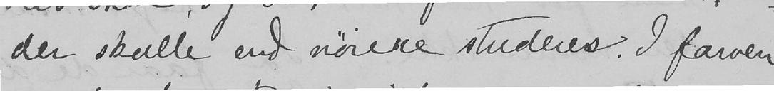der skulle end nøiere studeres. I farven
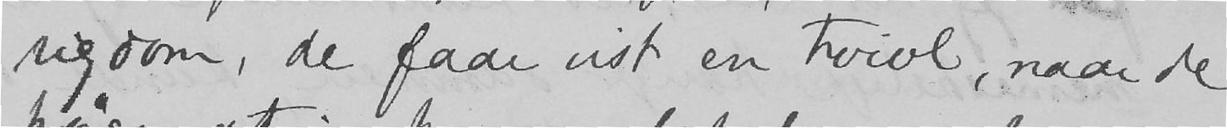rigdom, de faar vist en tvivl, naar de
